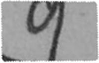9
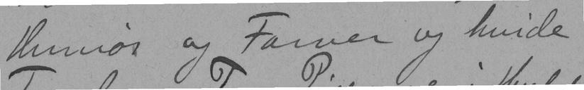Humør og Farver og hvide
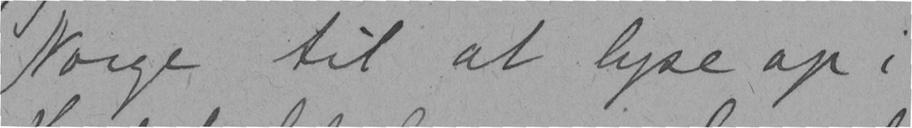Norge til at lyse op i
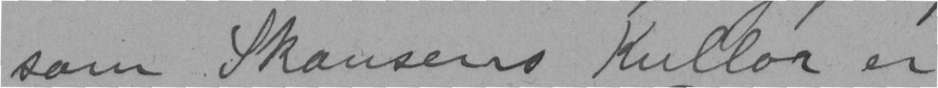som Skansens Kullor er
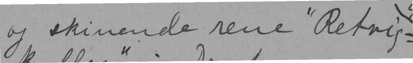og skinende rene "Retvig-
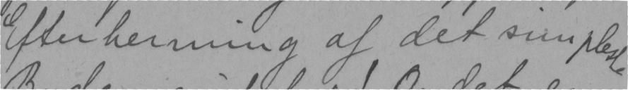Efterherming af det simpleste
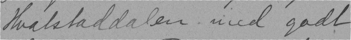Hvalstaddalen; med godt
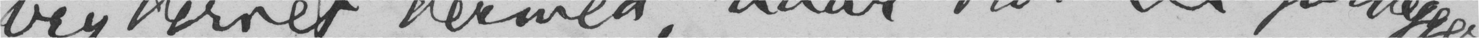bryderiet dermed, naar blot en forlægger
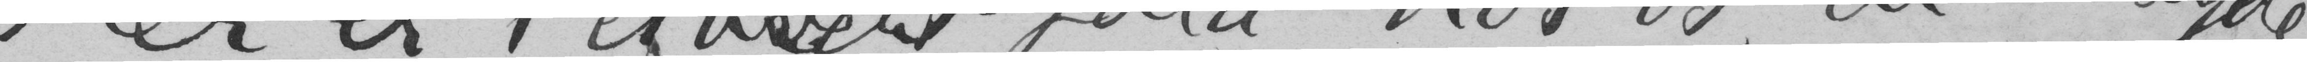Her er, i ethvert fald hos os, en mængde
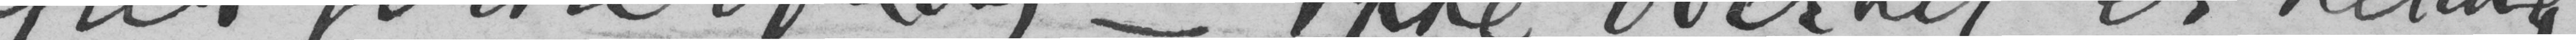efter første oplag - ikke overalt er heldig.
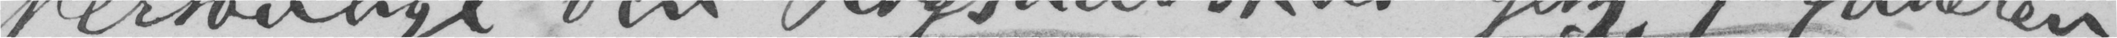personlige ven, Rigsadvokat Getz, forfatteren
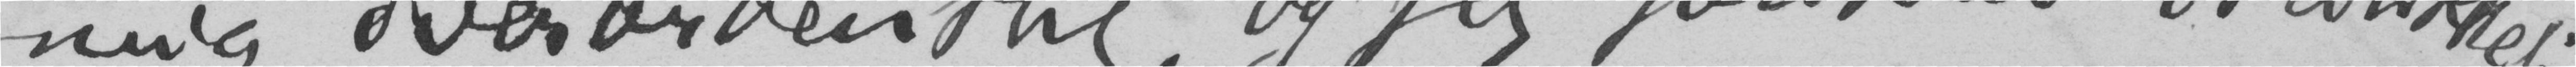mig overordentlig, og jeg forskrev øieblikkelig
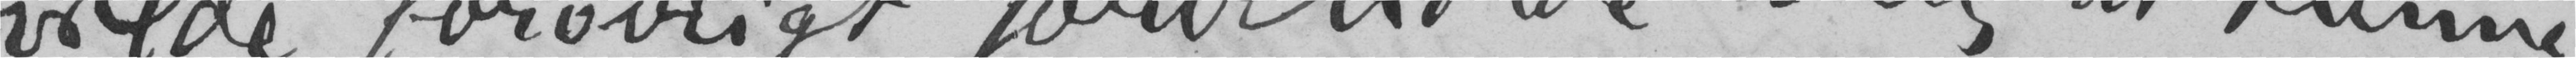vilde forøvrigt forbeholde mig at kunne
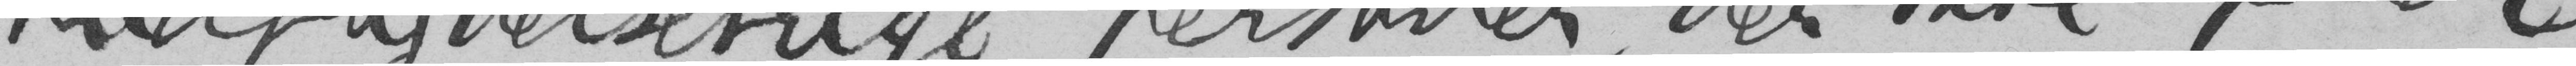indflydelsesrige personer, der ikke falde
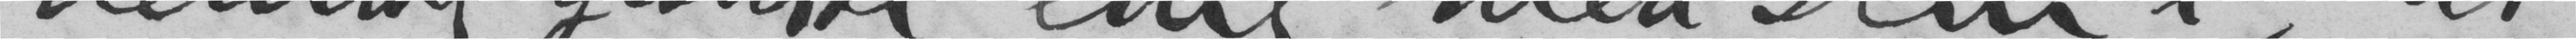nemlig ganske enig med Dem i, at
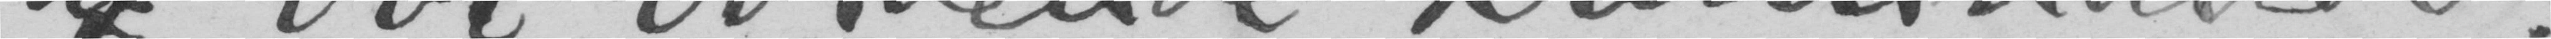af vor vordende kriminallov.
